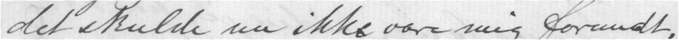det skulde nu ikke være mig forundt,
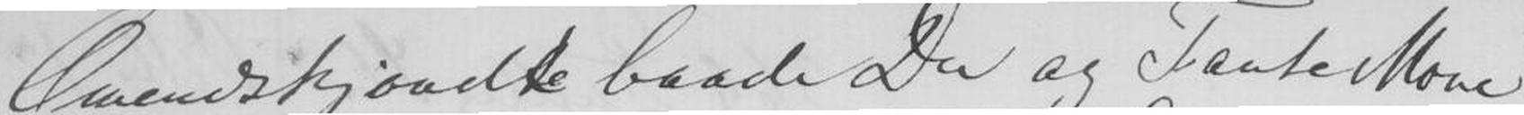Omendskjøndt baade Du og Tante Mone
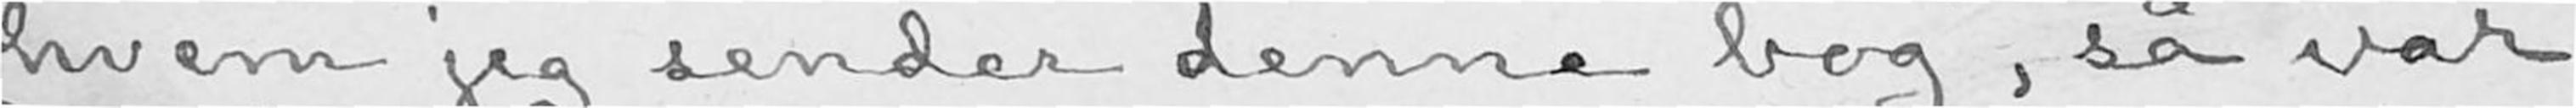hvem jeg sender denne bog, så var
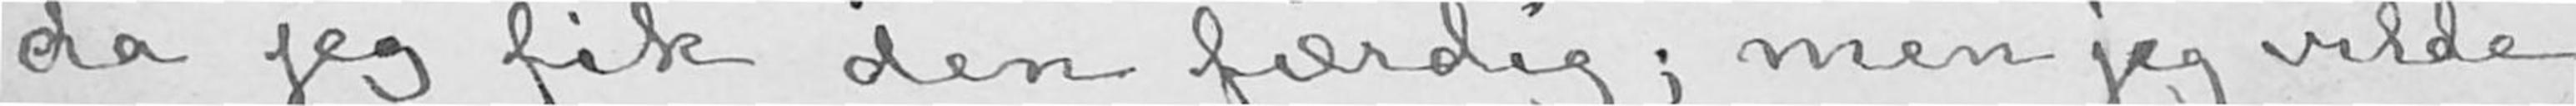da jeg fik den færdig; men jeg vilde
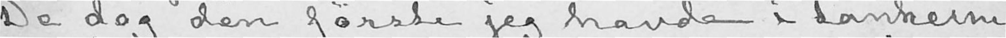De dog den første jeg havde i tankerne
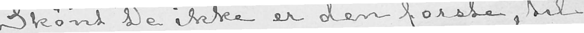Skønt De ikke er den første, til
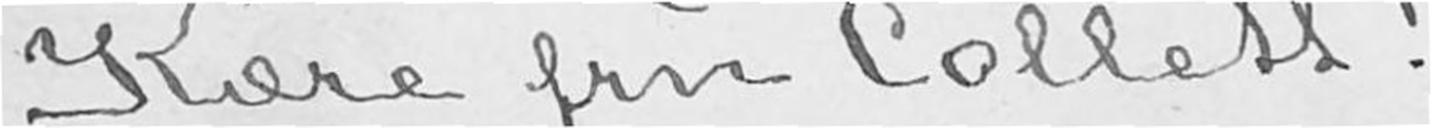Kære fru Collett!
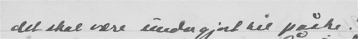det skal være undergjort til påske!
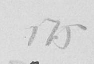175
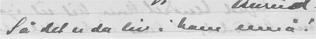Så det er da liv i ham ennå!
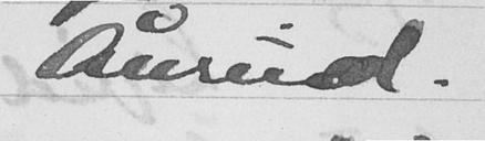Ånrud.
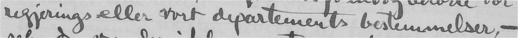regperings eller vort departements bestemmelser, -
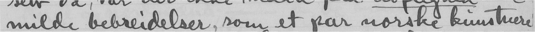milde bebreidelser, som et par norske kunstnere
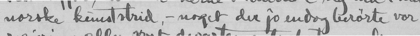norske kunststrid, - noget der jo endog berørte vor
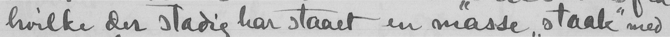hvilke der stadig har staaet en masse "staak" med
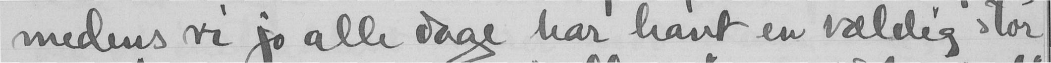medens vi jo alle dage har havt en vælelig stor
